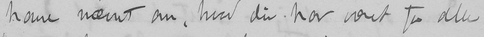have nævnt om, hvad du har været for alle
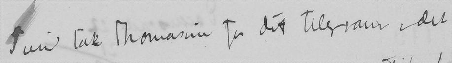Tusind tak Thomasine for dit telegram, det
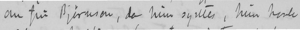om fru Bjørnson, da hun syntes, hun havde
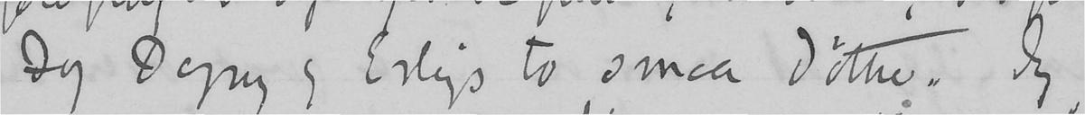Dog Dagny og Erlings to smaa døttre. Jeg
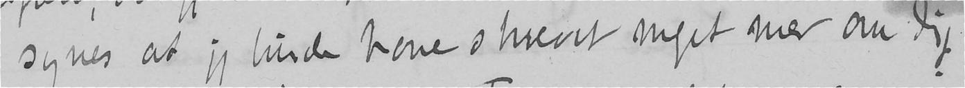synes, at jeg burde have skrevet meget mer om dig,
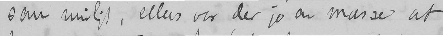som muligt, ellers var der jo en masse at
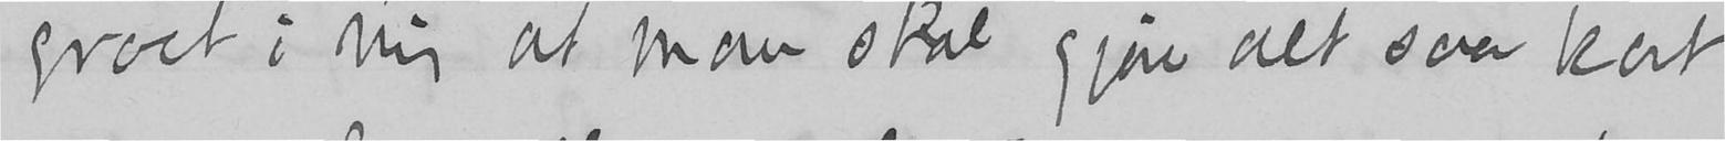groet i mig at man skal gjøre alt saa kort
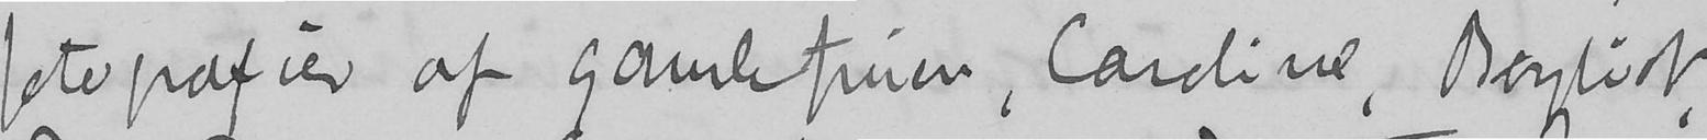fotografier af gamlefruen, Cardine, Bergliot,
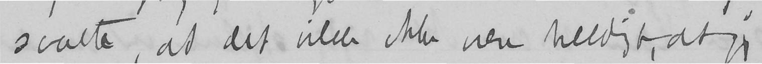svarte, at det vilde ikke være heldigt, at jeg
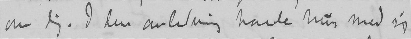om dig. I den anledning havde hun med sig
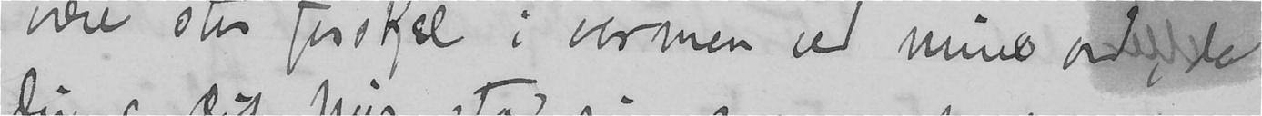være stor forskel i varmen ved mine ord, da
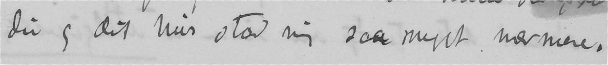du og dit hus stod mig saa meget nærmere,
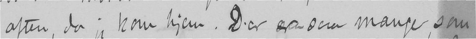aften, da kom hjem. Der er saa mange, som
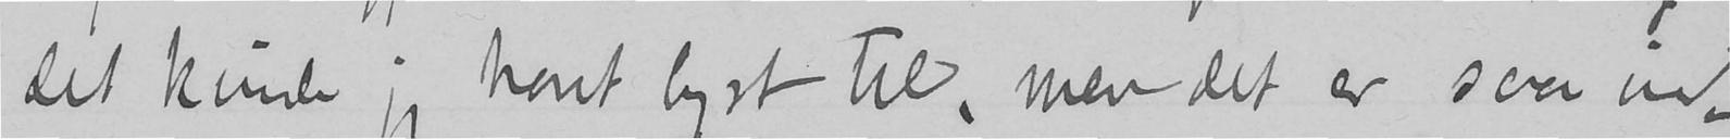det kunde jeg havt lyst til, men det er saa ind-
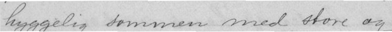hyggelig sammen med store og
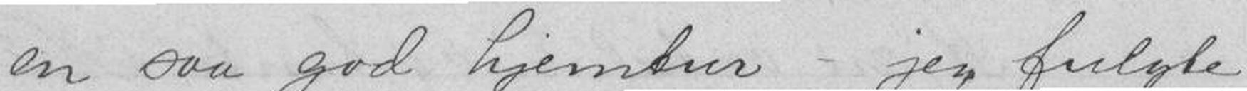en saa god hjemtur - jeg fulgte
